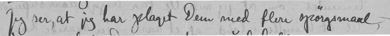Jeg ser, at jeg har plaget Dem med flere spørgsmaal, -
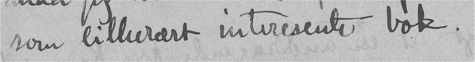som litterært interesente bok.
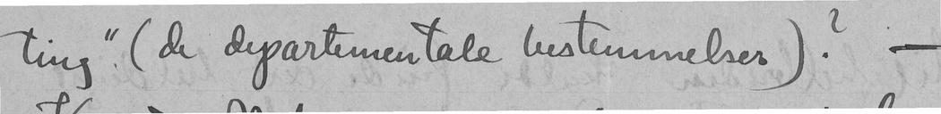ting". (de departementale bestemmelser)? -
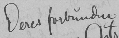Deres forbundne
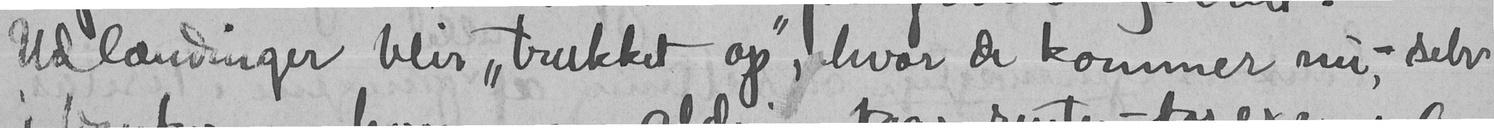udlændinger blir "trukket op", hvor de kommer nu, - selv
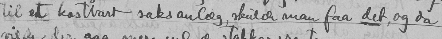til et kostbart saksanlæg, skulde man faa det, og da
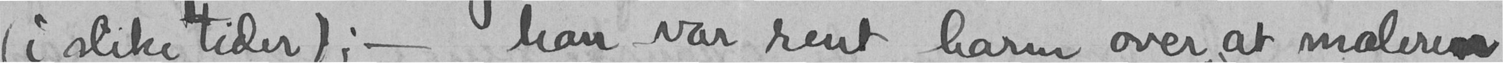(i slike tider); - han var rent harm over at maleren
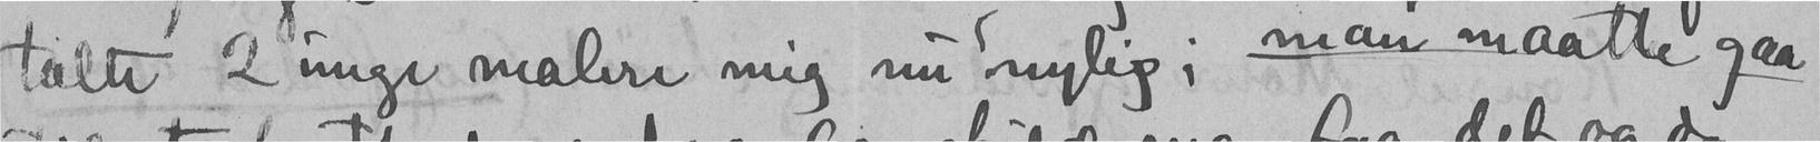talte 2 unge malere mig nu nylig; man maatte gaa
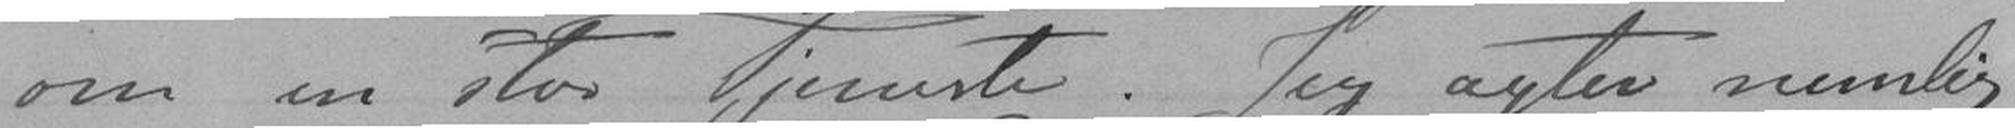om en stor Tjeneste. Jeg agter nemlig
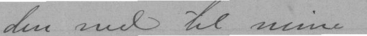den ned til mine
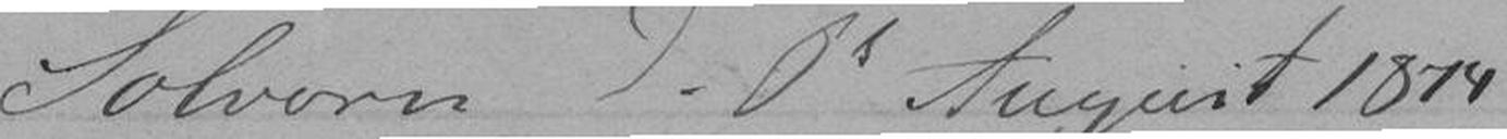Solvorn d.6t August 1874
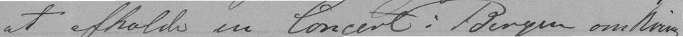at afholde en Concert i Bergen omkring
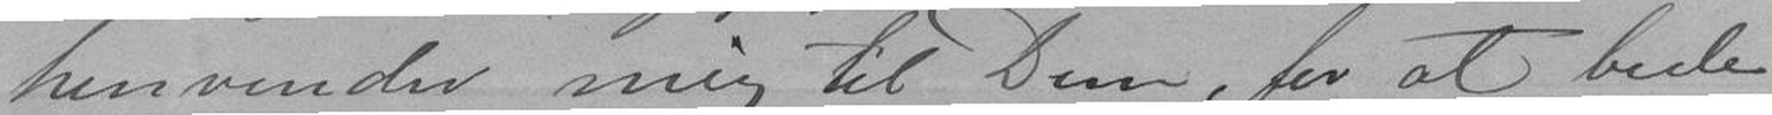henvender mig til Dem, for at bede
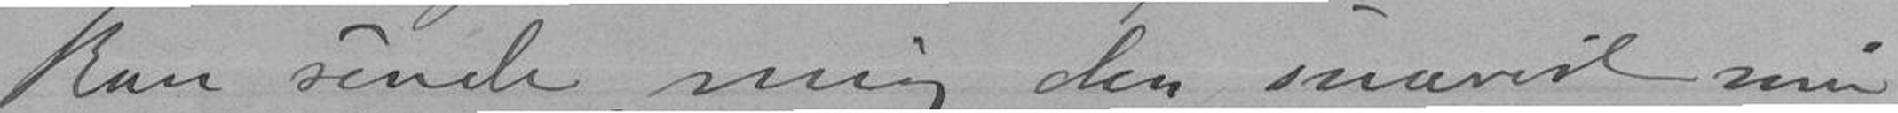kan sende mig den snarest mu-
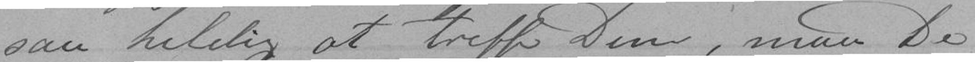saa heldig at treffe Dem, maa De
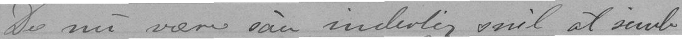De nu være saa indelig snil at sende
